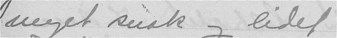meget snak og lidet
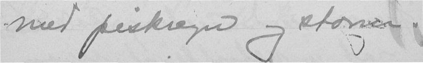med piskregn og storm.
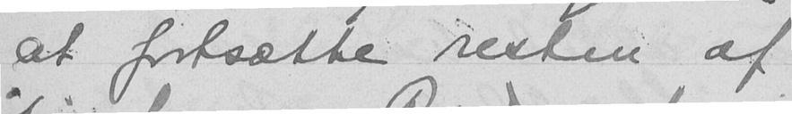at fortsætte resten af
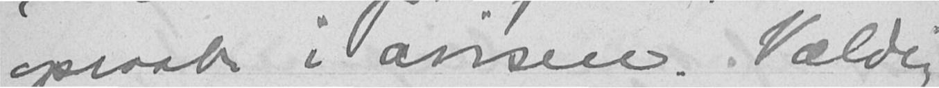opraab i avisen. Vældig
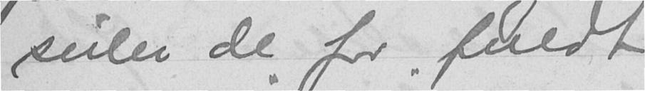seiler de for fuldt
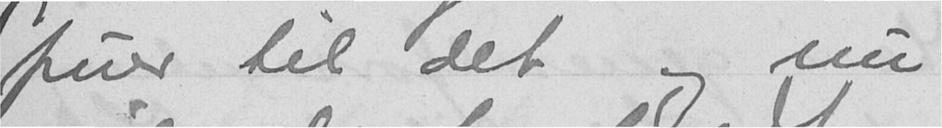fruer til det og nu
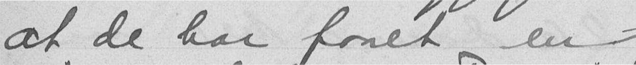at de har faaet en
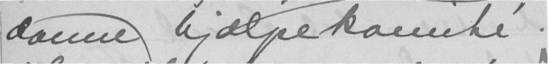denne hjælpekomité.
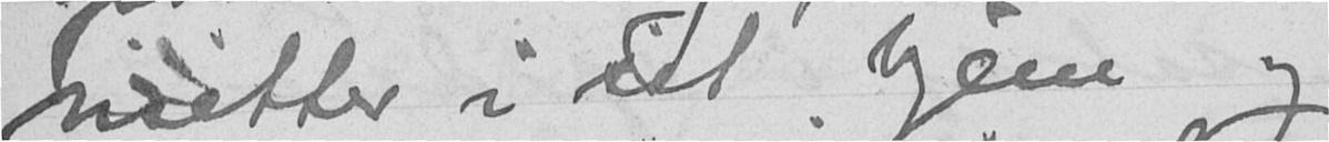visitter i sit hjem og
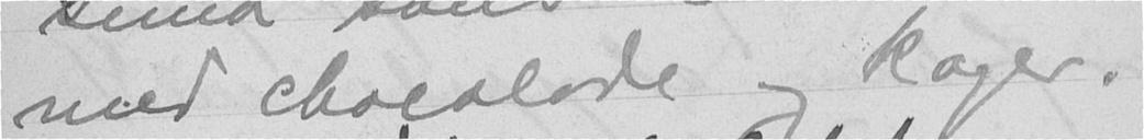med chocolade og kager.
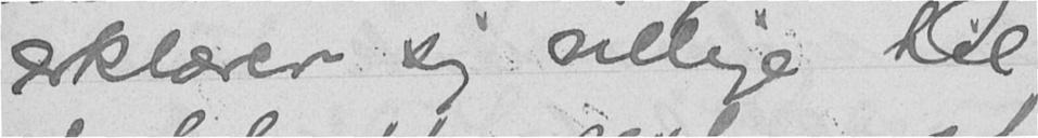erklærer sig villige til
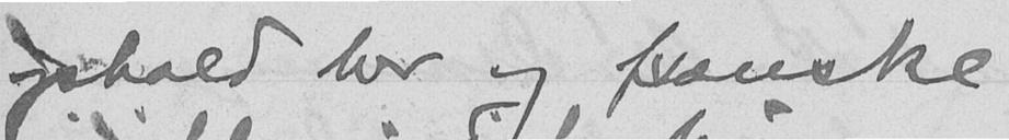ophold her og franske
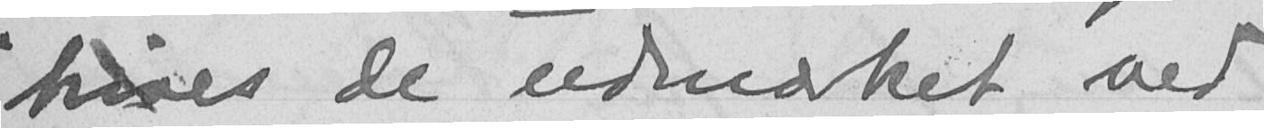trives de udmerket ved
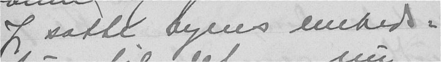Jeg satte byens embeds-
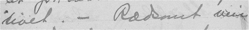livet. - Rædsomt veir
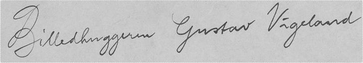Billedhuggeren Gustav Vigeland
